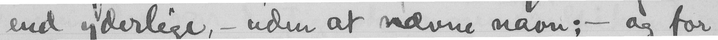end yderlige, - uden at nævne navn; - og for
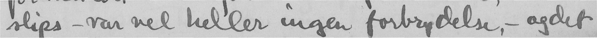slips - var vel heller ingen forbrydelse, - og det
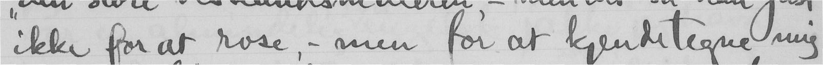ikke for at rose, - men for at kjendetegne mig
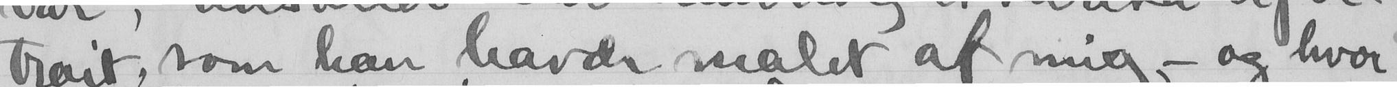trait, som han havde malet af mig, - og hvor
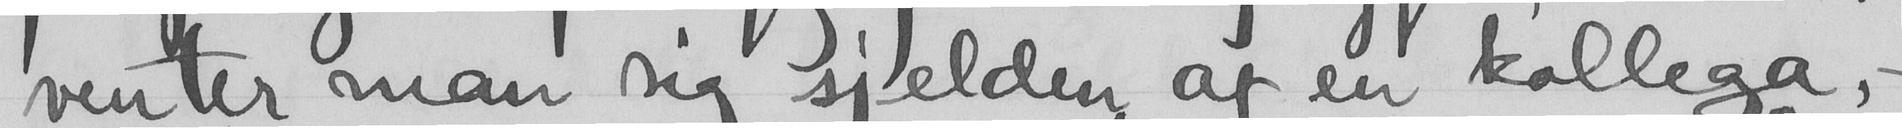venter man sig sjelden af en kollega,-
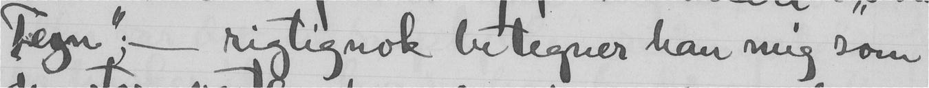Tegn; - rigtignok betegner han mig som
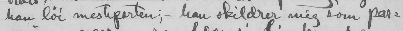han løi mesteparten; - han skildrer mig som par-
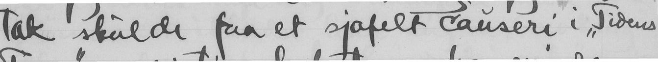tak skulde faa et sjofelt causeri i "Tidens
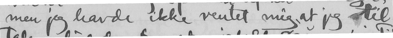men jeg havde ikke ventet mig at jeg til
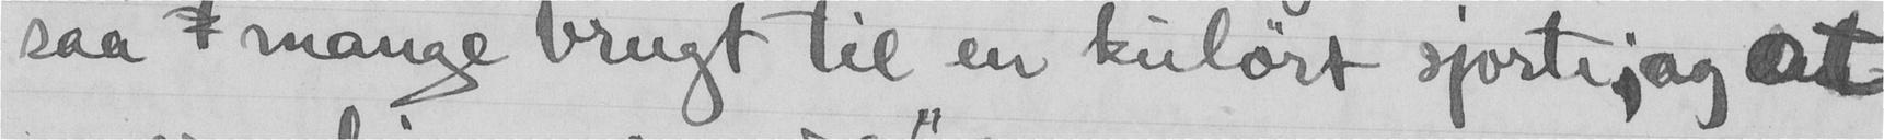saa  mange brugt til en kulørt sjorte; og at
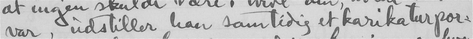var, udstiller han samtidig et Karikaturpor-
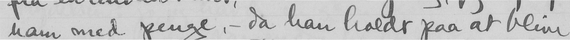ham med penge, - da han holdt paa at blive
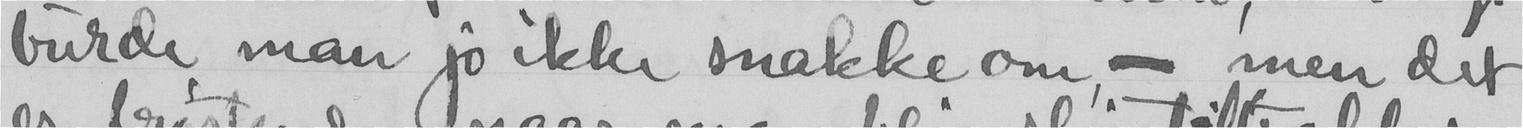burde man jo ikke snakke om - men det
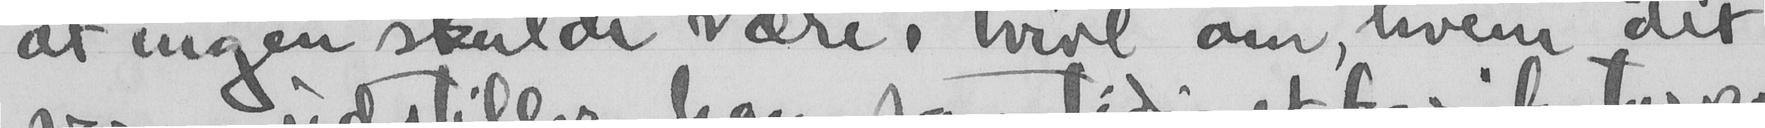at ingen skulde være i tvivl om, hvem det
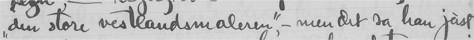"den store vestlandsmaleren" - men det sa han just
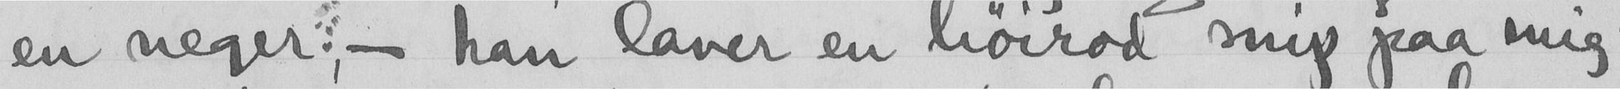en neger; - han laver en høirod snip paa mig
